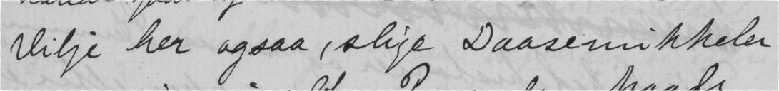vilje her ogsaa, slige Daasemikkeler
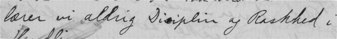lærer vi aldrig Disiplin og Raskhed i
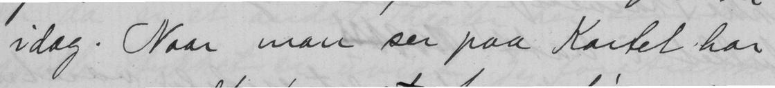idag. Naar man ser paa Kartet har
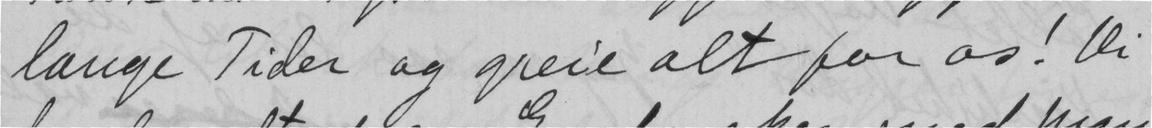lange Tider og greie alt for os! Vi
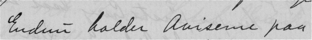Endnu holder Aviserne paa
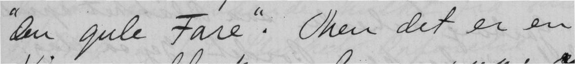"den gule Fare". Men det er en
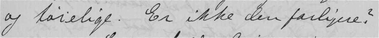og tøielige. Er ikke den farligere?
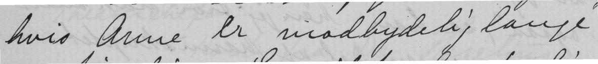hvis Arme er modbydelig lange
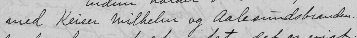med Keiser Wilhelm og Aalesundsbranden.
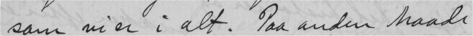som vi er i alt. Paa anden Maade
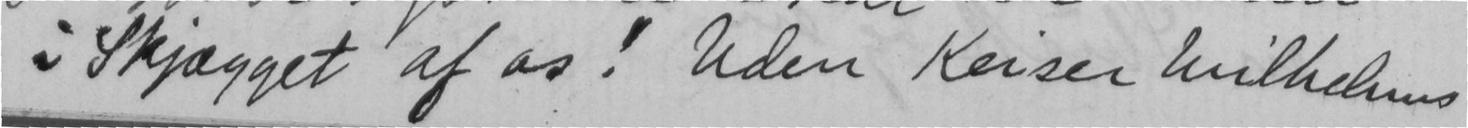i Skjægget af os! Uden Keiser Wilhelms
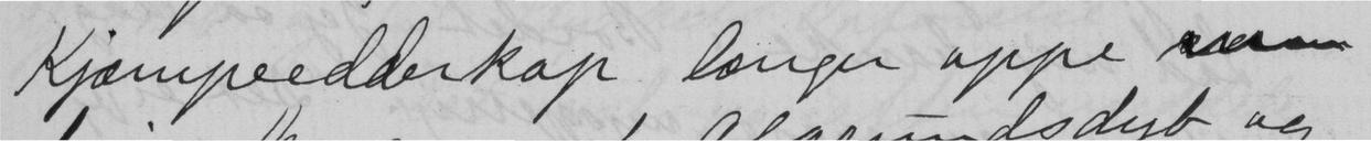Kjæmpeedderkop længer oppe sam
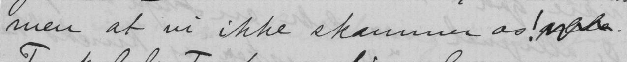men at vi ikke skammer os!
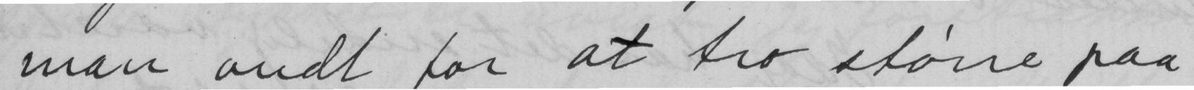man ondt for at tro større paa
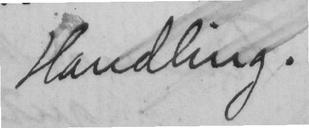Handling.
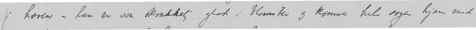i haven – han er saa skrækkelig glad i blomster og kommer hele dagen hjem med
